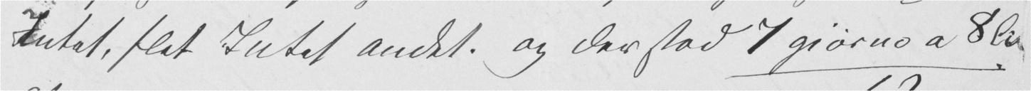Intet, slet Intet andet. og der stod 7 giorno a 8 Li
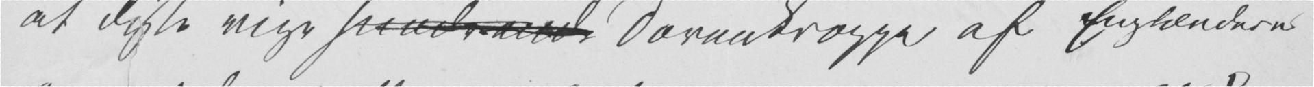at disse rige hundrende Darmkroppe af Englænderne
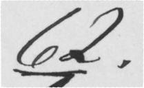62.
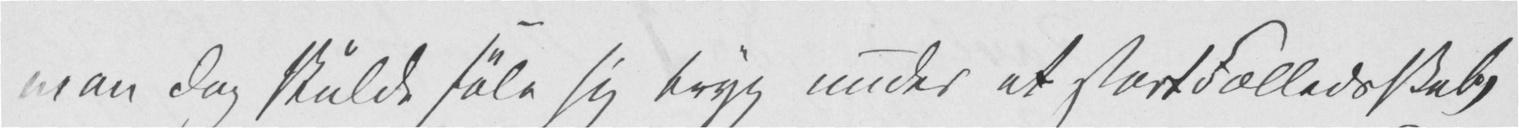man dog skulde føle sig tryg under et stort Fælledsskab,
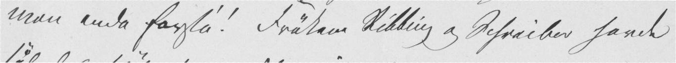man enda forstå! Frøken Ribbing og Schreiber havde
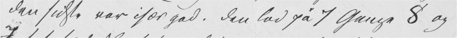Den sidste var især god. Den lød på 7 Gange 8 og
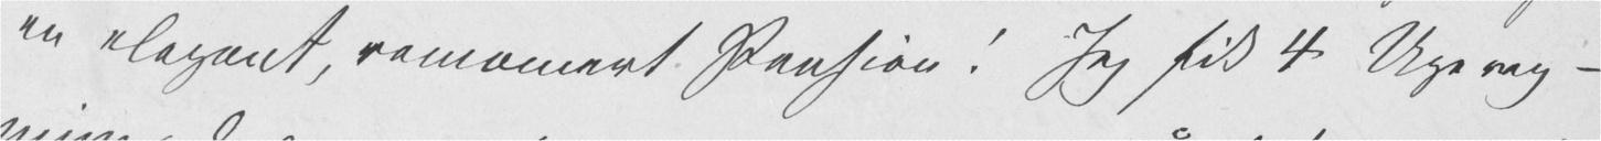en elegant, remomert Pension! Jeg fik 4 Ugereg-
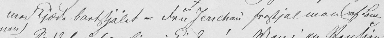med Kjæde bortstjålet – Fru Jerichau frastjal man (af lom-
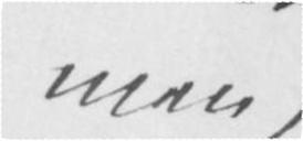men
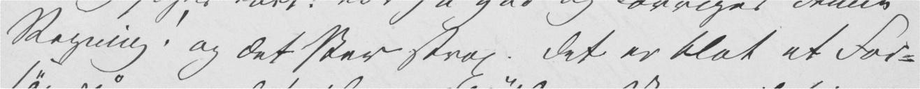Regning! og det sker strax. Det er blot et For-
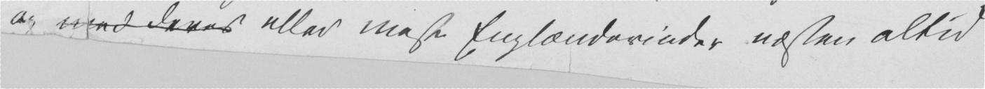og med deres eller meste Englænderinder næsten altid
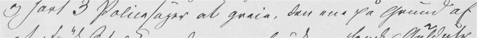og havt 3 Policesager at greie, den ene på Grund af
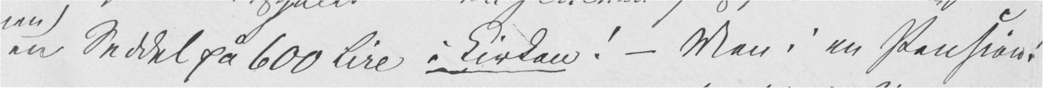en Seddel på 600 Lire i Kirken! – Men i en Pension!
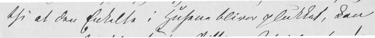thi at den Enkelte i Husene bliver plukket, kan
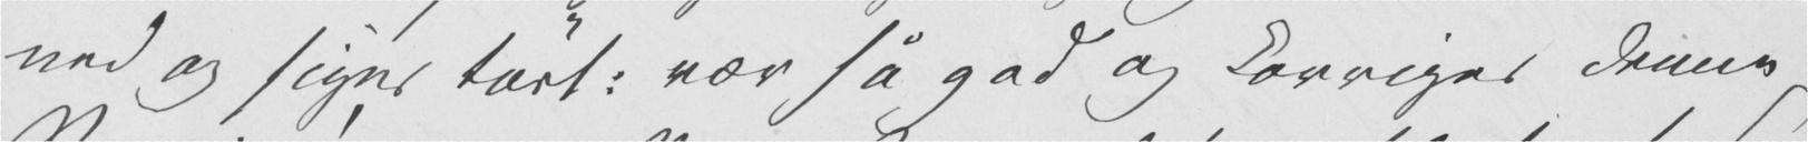ned og siger tørt: vær så god og korriger denne
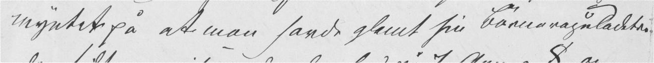myntet på at man havde glemt sin Børnevexeladets.
